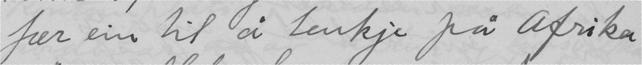fer ein til å tenkje på Afrika
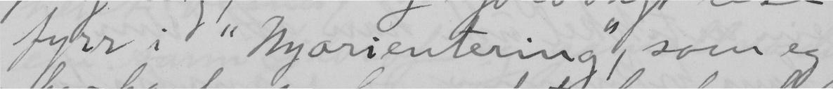fyrr i "Nyorientering", som eg
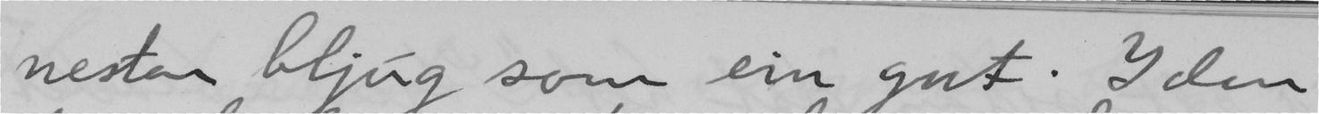nesten bljug som ein gut. I den
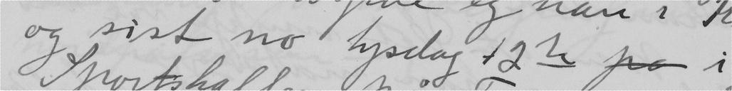og sist no tysdag 12te i
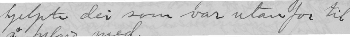hjelpte dei som var utanfor til
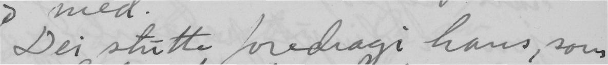Dei stutte foredragi hans, som
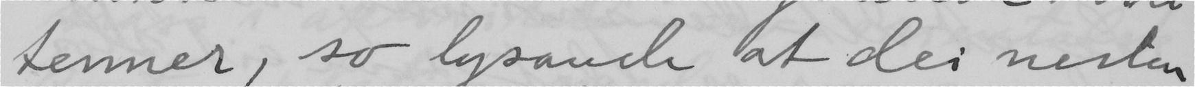tenner, so lysande at dei nesten
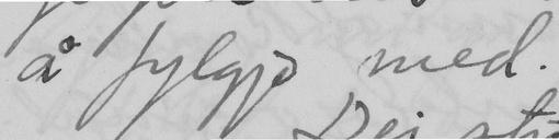å fylgjo med.
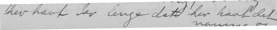hev havt so lenge det hev havt det
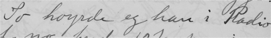So höøyrde eg han i Radio
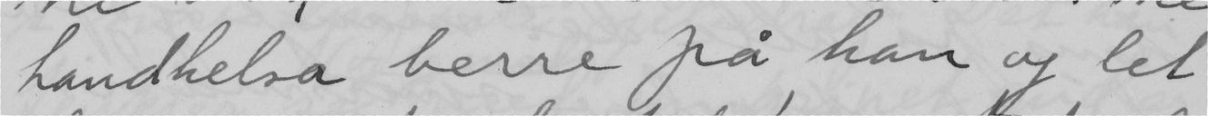handhelsa berre på han og let
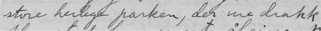store herlege parken, der me drakk
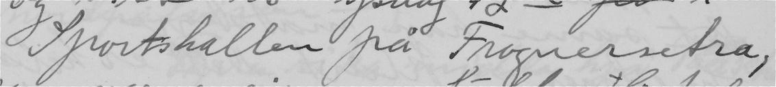Sportshallen på Frognersetra,
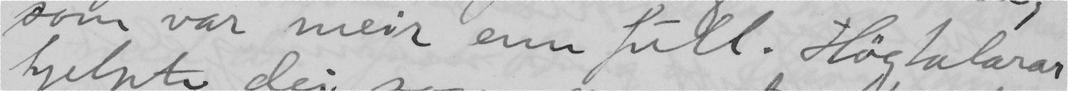som var meir enn full. Högtalarar
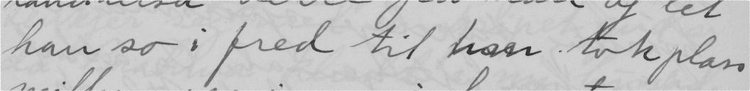han so i fred til han tok plass
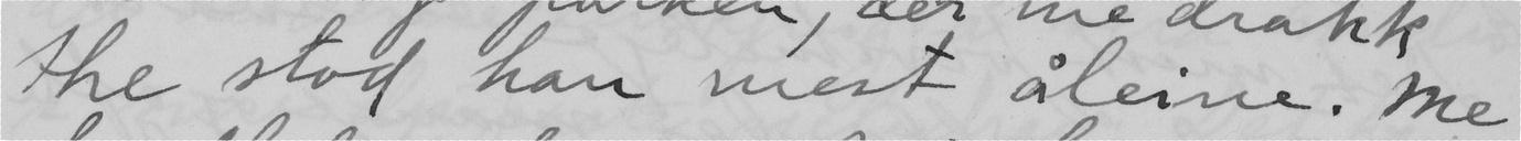the stod han mest åleine. Me
